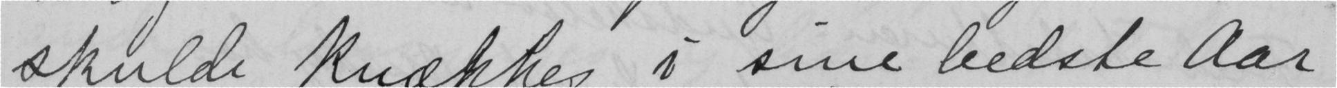skulde knækkes i sine bedste Aar
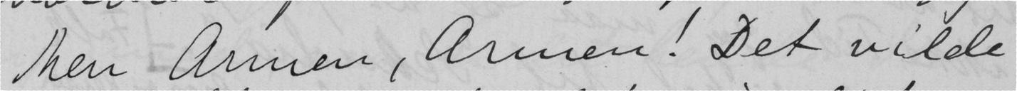Men armen, armen! Det vilde
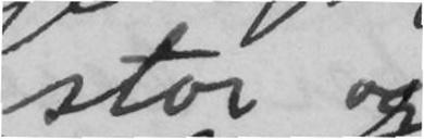stor og
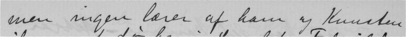men ingen lærer af ham og Kunsten
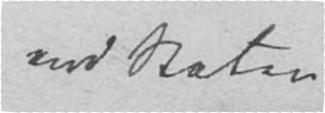end Staten
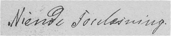Niende Forelæsning.
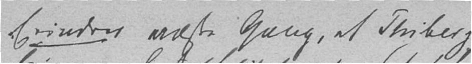Erindres næste Gang, at Thiberg-
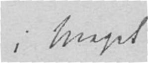i Meget
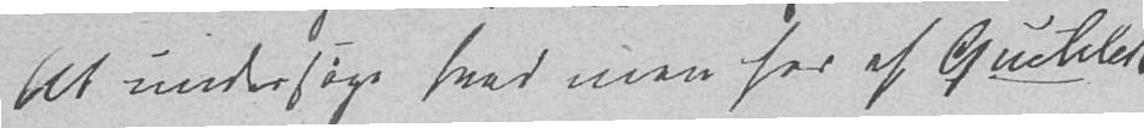At undersøge hvad man har af Gubelet
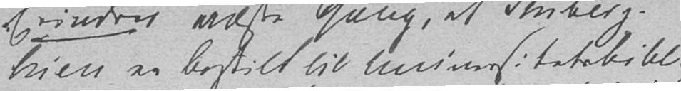hien er bestilt til Universitetsbibl.
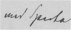med Sparta
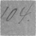104.
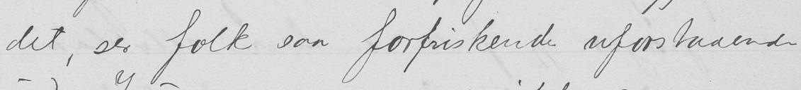det, ser folk saa forfriskende uforstaaende
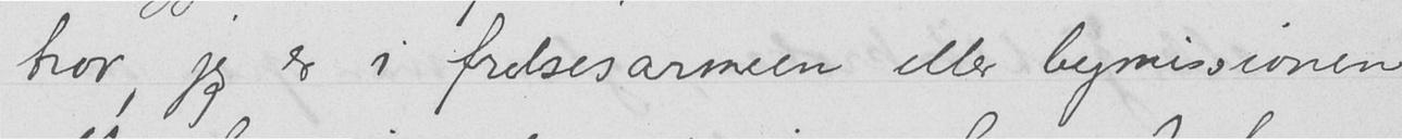tror, jeg er i frelsesarmeen eller bymissionen
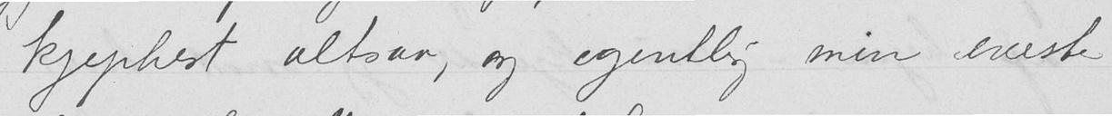kjephest altsaa, og egentlig min eneste
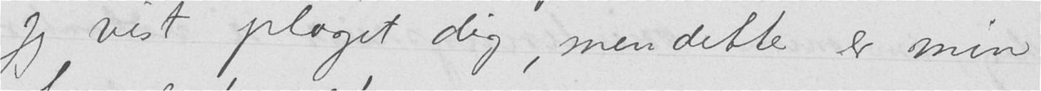jeg vist plaget dig, men dette er min
